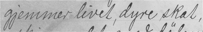gjemmer livet, dyre skat,
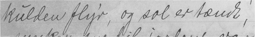kulden flyr, og sol er tændt,
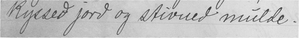kyssed jord og stivned mulde.
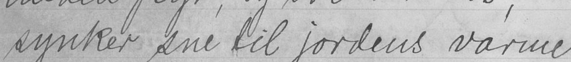synker sne til jordens varme
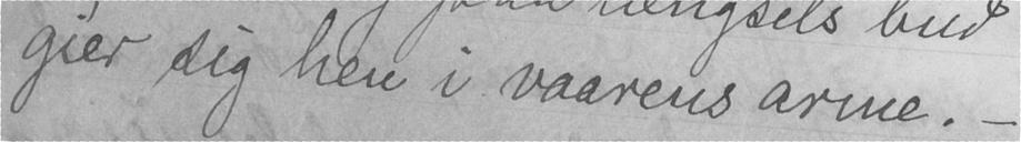gi'er sig hen i vaarens arme. -
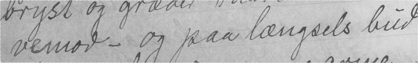vemod - og paa længsels bud
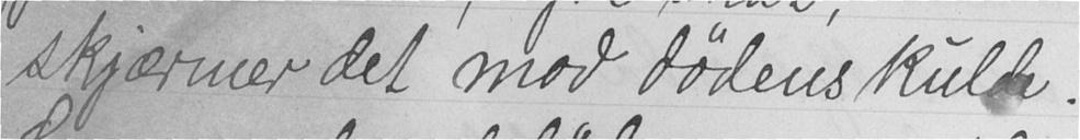skjærmer det mod dødens kulde.
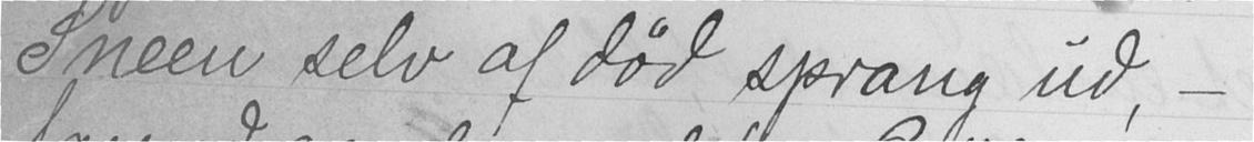Sneen selv af død sprang ud, -
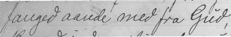fanged aande med fra Gud,
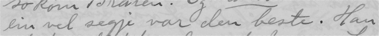ein vel segje var den beste. Han
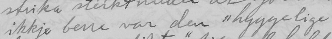ikkje berre var den "hyggelige
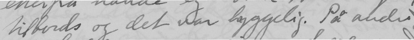tilbords og det var hyggelig. På andre
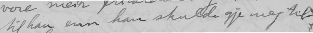til han enn han skulde gje meg til
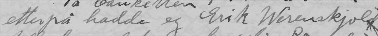etterpå hadde eg Erik Werenskjold
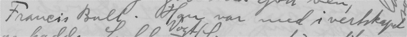Francis Bull. Han var med i vertskapet
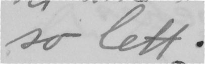so lett.
